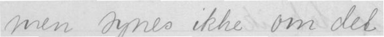men synes ikke om det
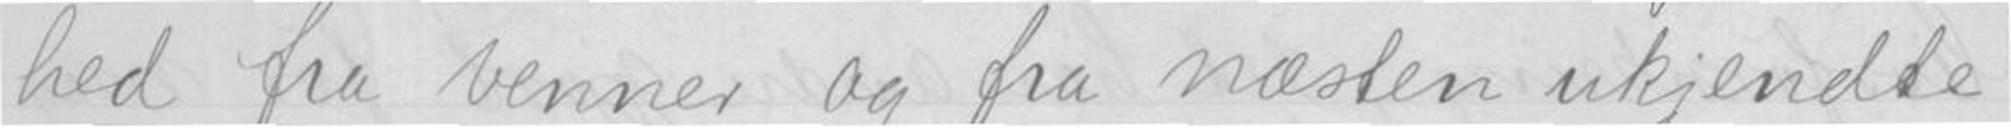hed fra venner og fra næsten ukjendte
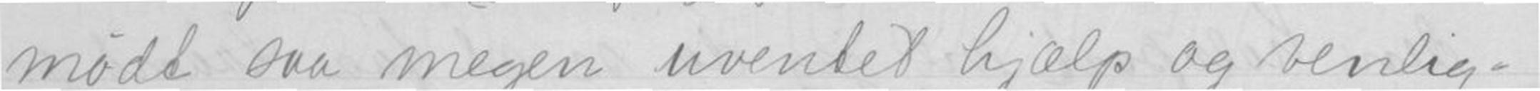mødt saa megen uventet hjælp og venlig-
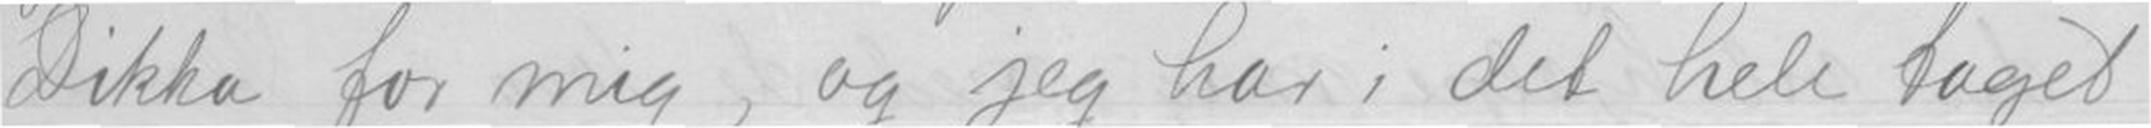Dikka for mig, og jeg har i det hele taget
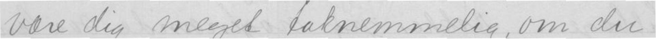være dig meget teknemmelig, om du
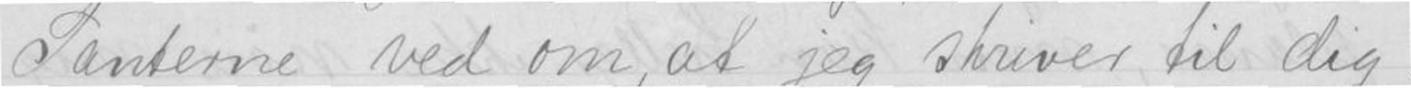- Tanterne ved om at jeg skriver til dig,
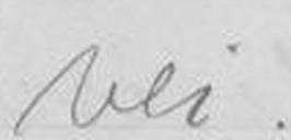vei.
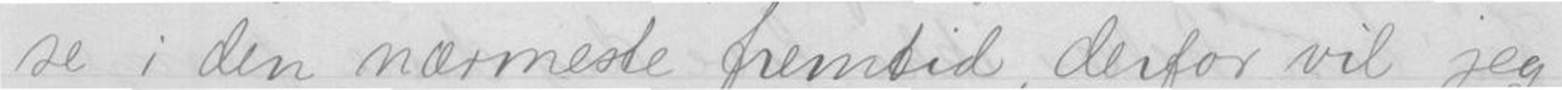se i den nærmeste fremtid, derfor vil jeg
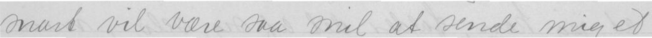snart vil være saa snil at sende mig et
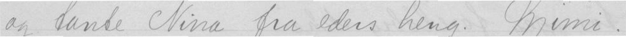og tante Nina fra eders heng. Mimi.
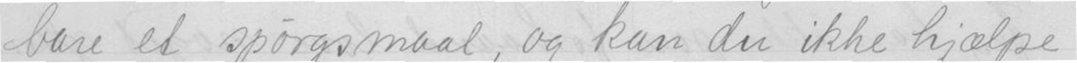bare et spørgsmaal, og kan du ikke hjælpe
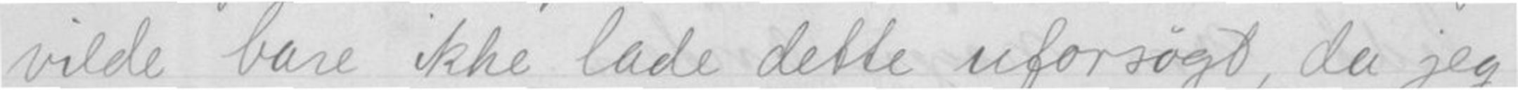vilde bare ikke lade dette uforsøgt, da jeg
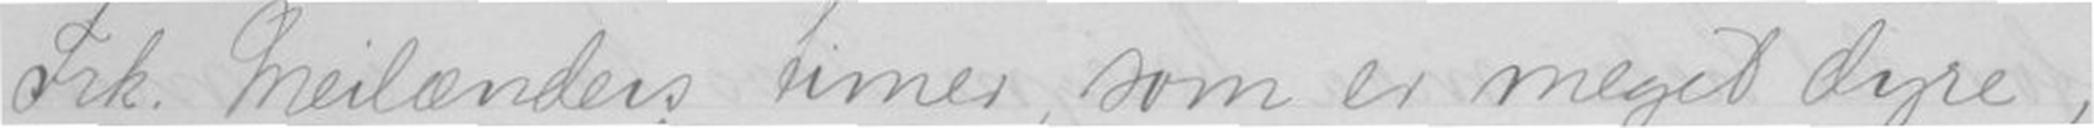Frk Meilænders timer, som er meget dyre,
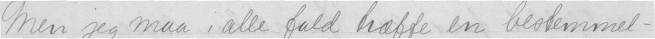Men jeg maa i alle fald træffe en bestemmel-
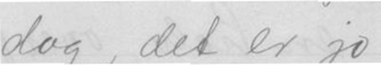dog, det er jo
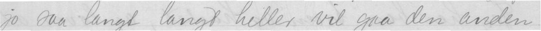jo saa langt langt heller vil gaa den anden
